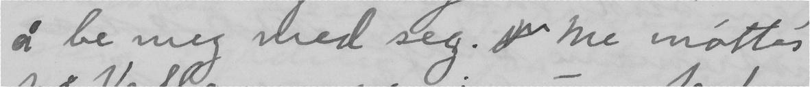å be meg med sig.  Me möttes
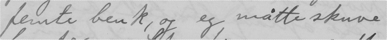femte benk, og eg måtte skuve
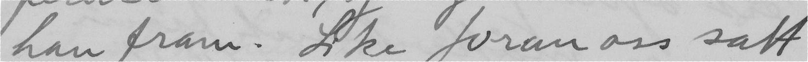han fram. Like foran oss satt
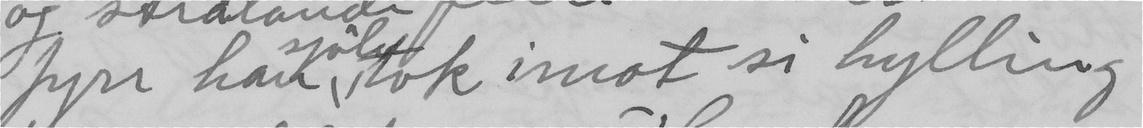fyrr han sjölv tok imot si hylling
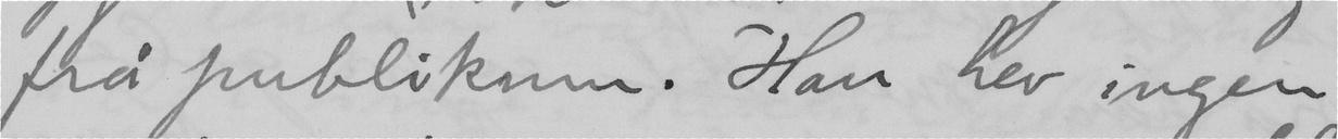frå publikum. Han hev ingen
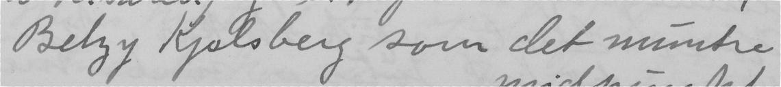Betzy Kjelsberg som det muntre
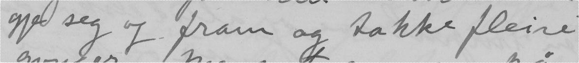gje seg og fram og takke fleire
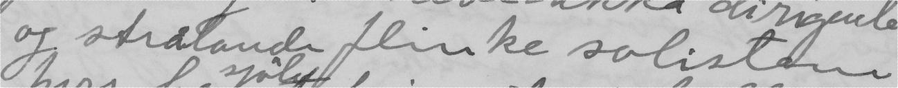og strålande flinke solistene
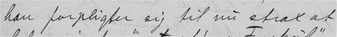han forpligter sig til nu strax at
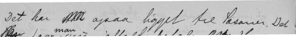Det har  ogsaa ligget tre Pasaner. Det
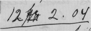12  2.04
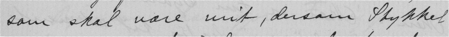som skal være mit, dersom Stykket
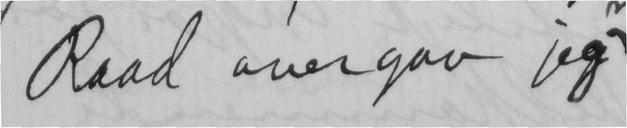Raad overgav jeg
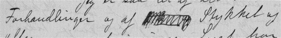Forhandlinger og af  Stykket og
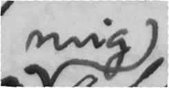mig
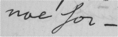noe for -
